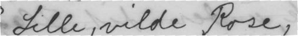Lille, vilde Rose,
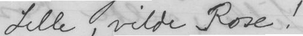Lille, vilde Rose!
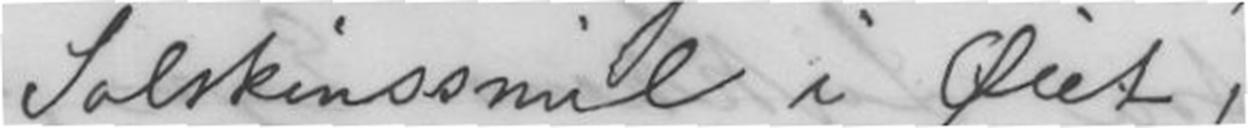Solskinssmil i Øiet,
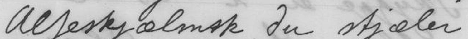Alfeskjælmsk du stjæler
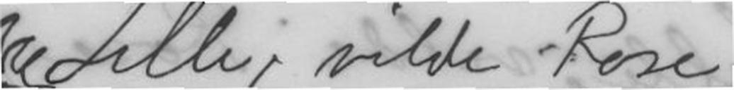Lille, vilde Rose
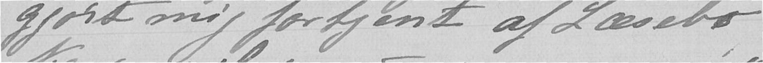gjort mig fortjent af Læsebo-
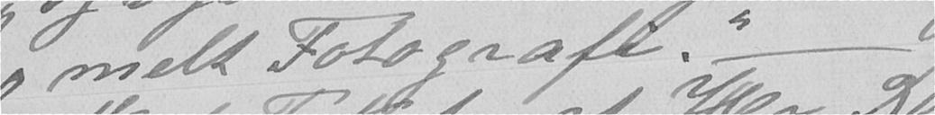"melt Fotografi." -
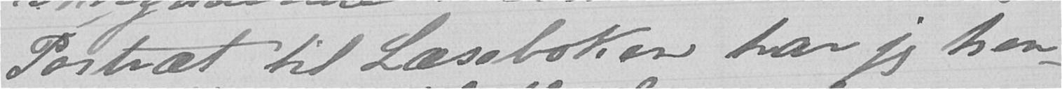Portræt til Læseboken har jeg hen-
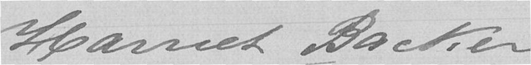Harriet Backer
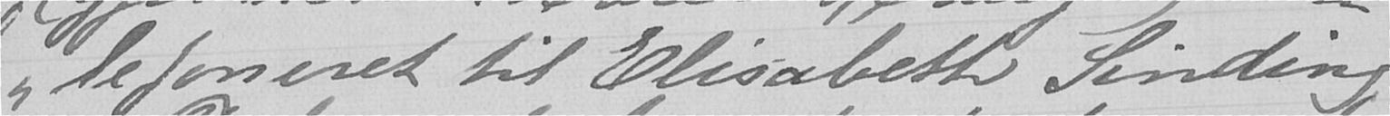"lefoneret til Elisabeth Sinding,
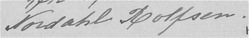Nordahl Rolfsen.
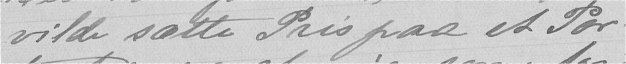vilde sætte Pris paa et Por-
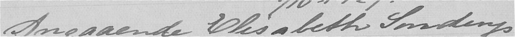Angaaende Elisabeth Sindings
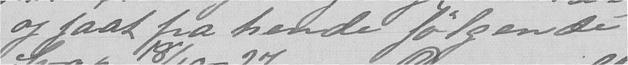og faat fra hende følgende
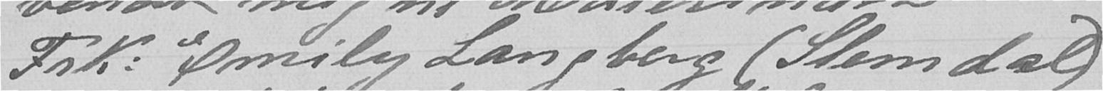Frk. Emily Langberg (Slemdal)
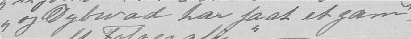"og Dybwad har faat et gam-
